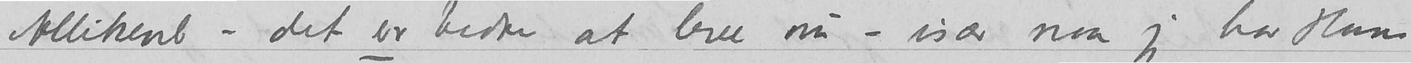Allikevel – det er bedre at leve nu – især naar jeg har Hans
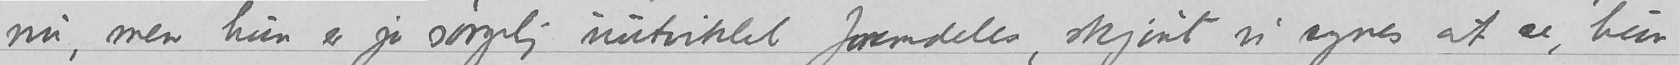nu, men hun er jo sørgelig uutviklet fremdeles, skjønt vi synes at se, hun
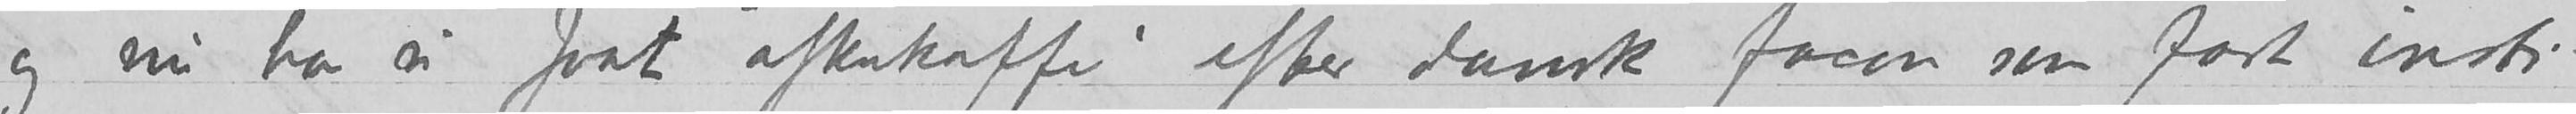og nu har vi faat «aftenkaffe» efter dansk facon som fast insti-
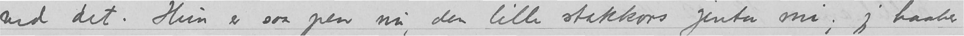ved det. Hun er saa pen nu, den lille stakkars jenta mi; jeg haaber
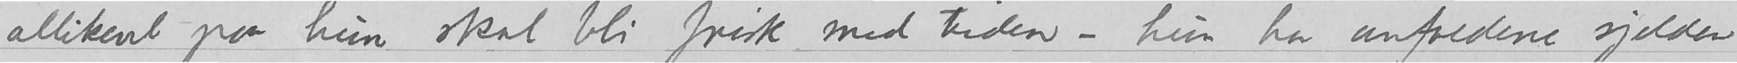allikvel paa hun skal bli frisk med tiden – hun har anfaldene sjelden
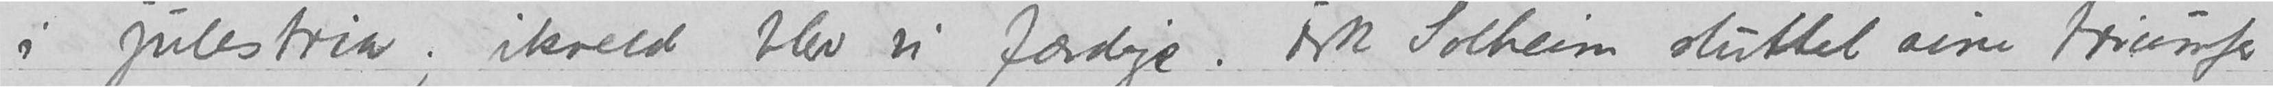i julestria, ikveld blev vi ferdige.  Frk Solheim sluttet sine triumfer
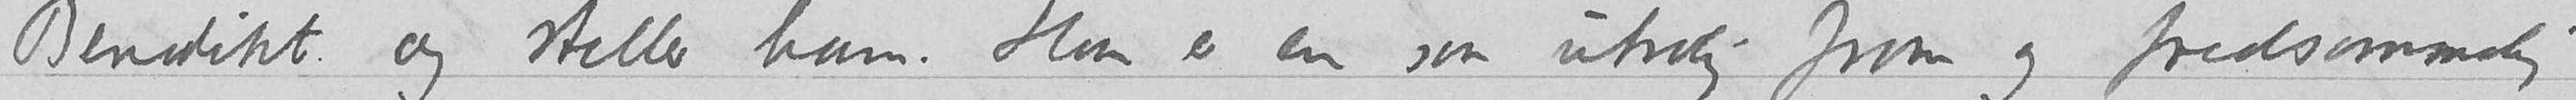Benedikt og steller ham. Han er en saa utrolig from og fredsommelig
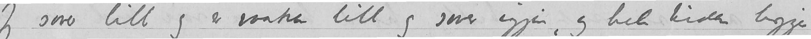Jeg sover litt og er vaaken litt og sover igjen, og hele tiden ligger
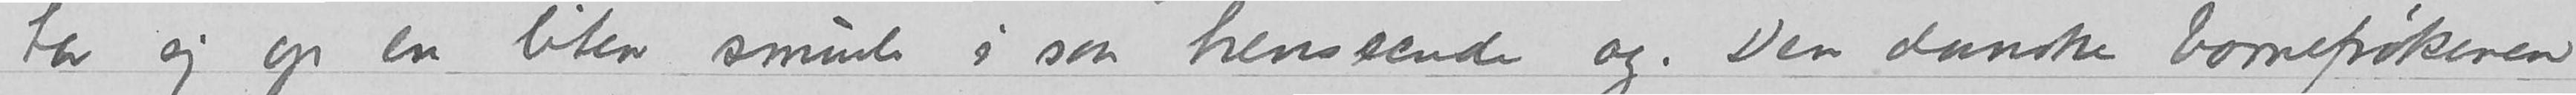tar sig op en liten smule i saa henseende og. Den danske barnefrøkenen
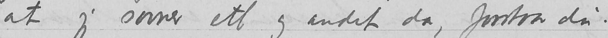at jeg savner ett og andet da, forstaar du.
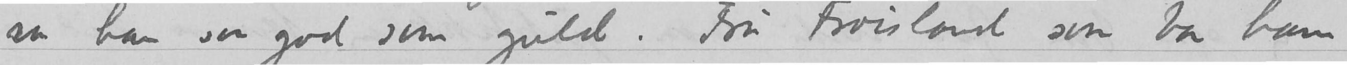var han saa god som guld. Fru Frøisland som bar ham
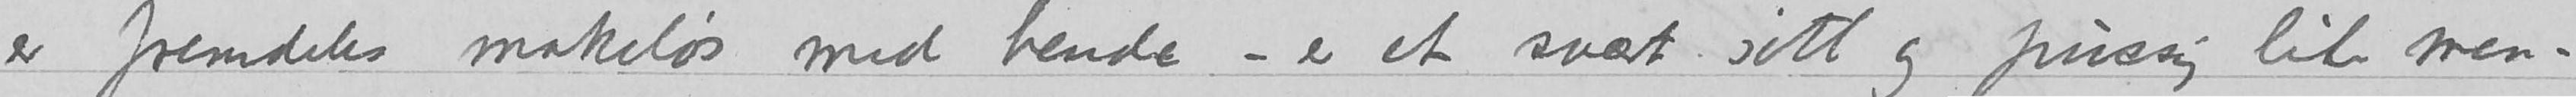er fremdeles makeløs med hende – er et svært søtt og pussig lite men-
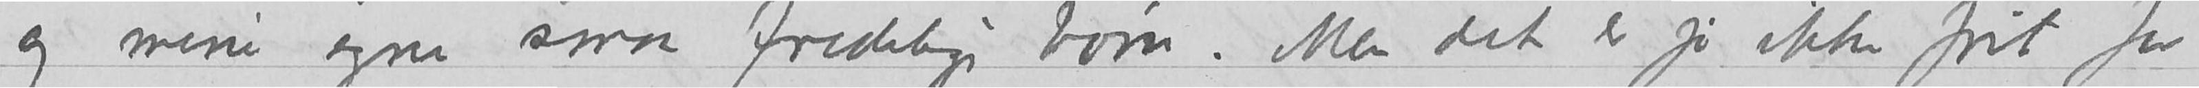og mine egne smaa fredelige børn. Men det er jo ikke frit for
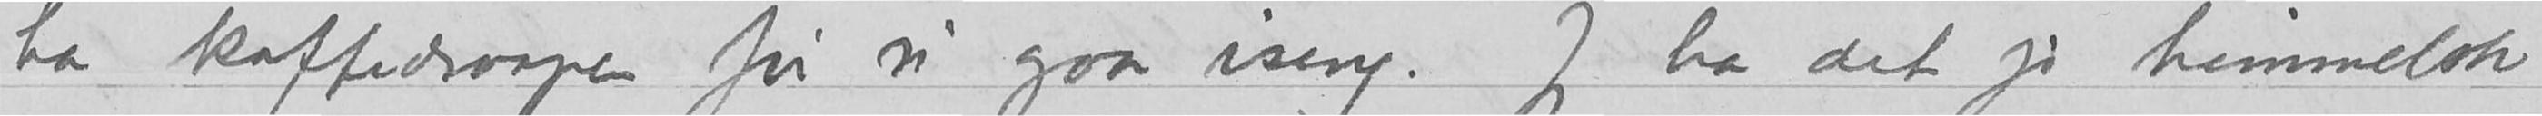ha kaffedraapen før vi gaar iseng. Jeg har det jo himmelsk
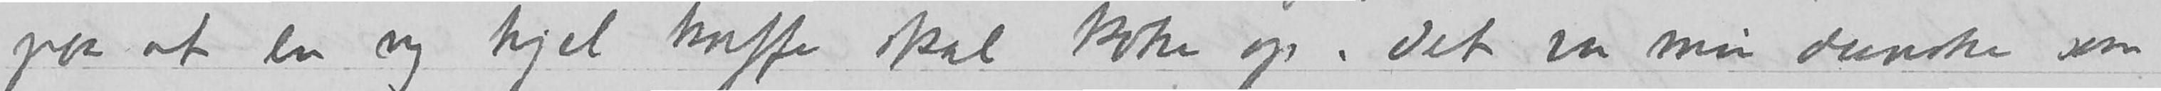paa at en ny kjel kaffe skal koke op. Det var min danske som
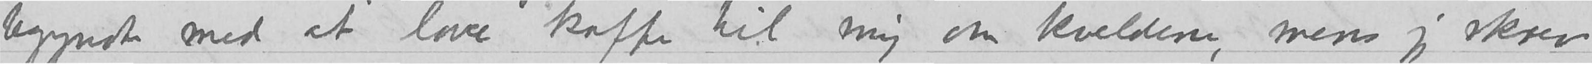begyndte med at lave kaffe til mig om kveldene, mens jeg skrev
